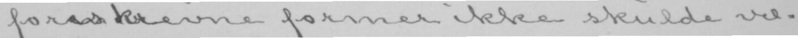formeskrevne former ikke skulde væ-
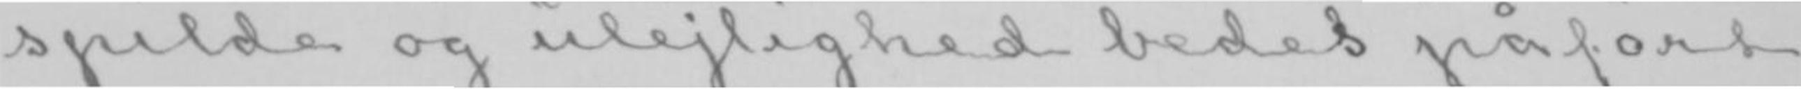spilde og ulejlighed bedes påført
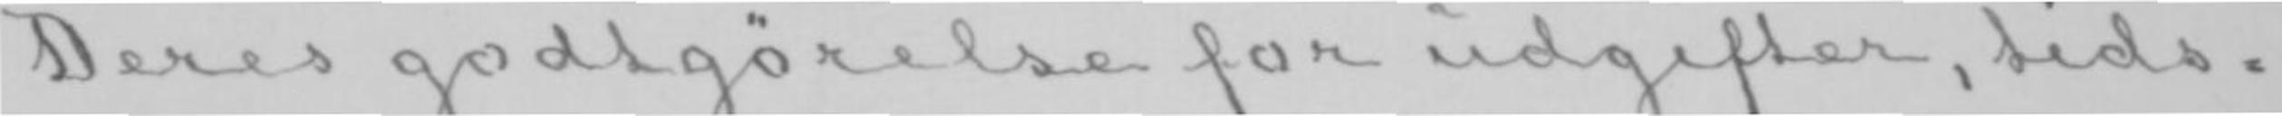Deres godtgørelse for udgifter, tids-
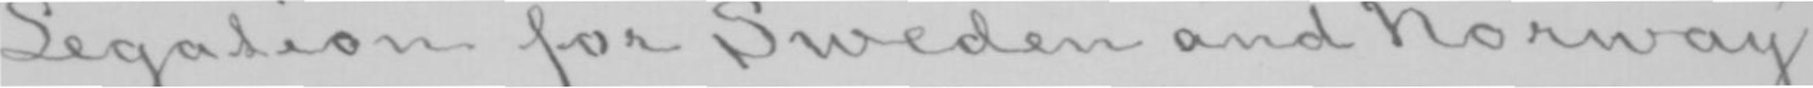Legation for Sweden and Norway.
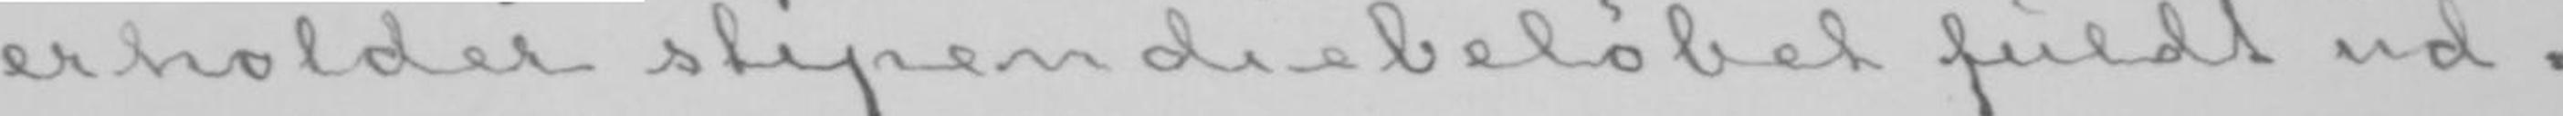erholder stipendiebeløbet fuldt ud-
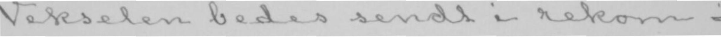Vekselen bedes sendt i rekom-
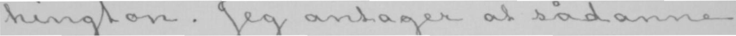hington. Jeg antager at sådanne
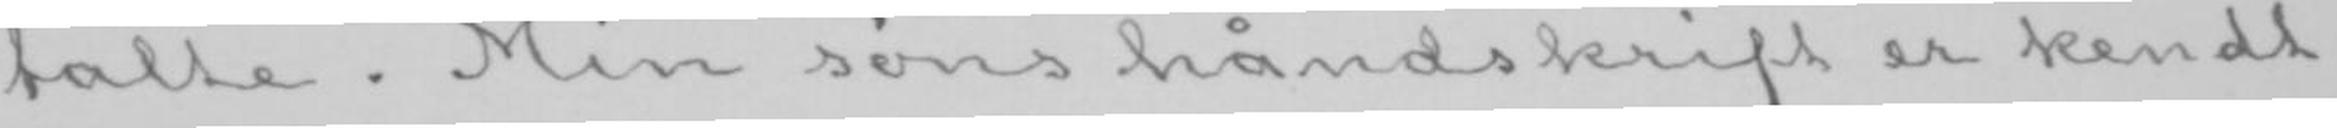talte. Min søns håndskrift er kendt
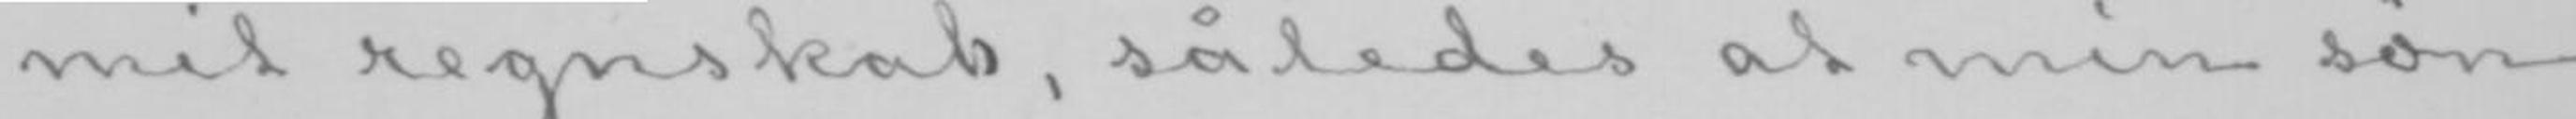mit regnskab, således at min søn
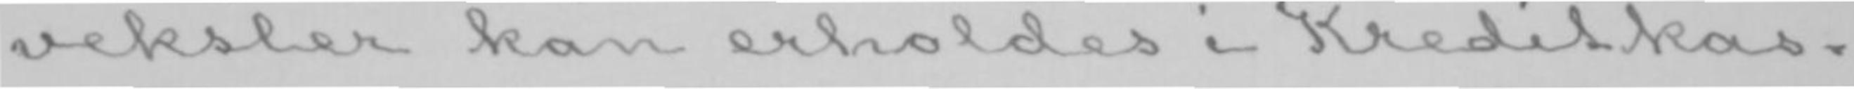veksler kan erholdes i Kreditkas-
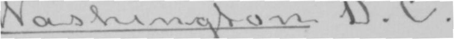Washington D. C.
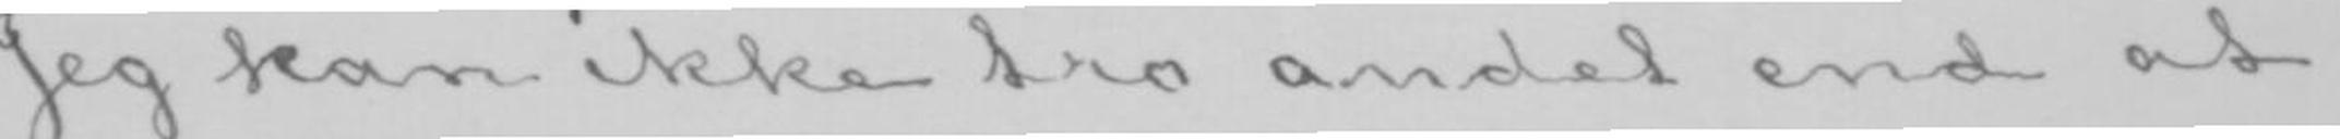Jeg kan ikke tro andet end at
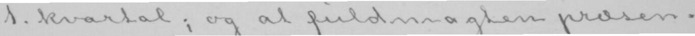1. kvartal; og at fuldmagten præsen-
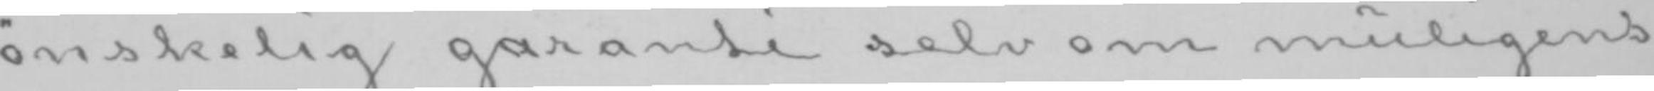ønskelig garanti selv om muligens
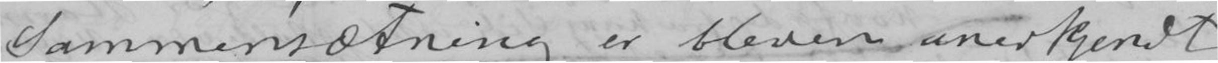Sammensætning er bleven anerkjendt
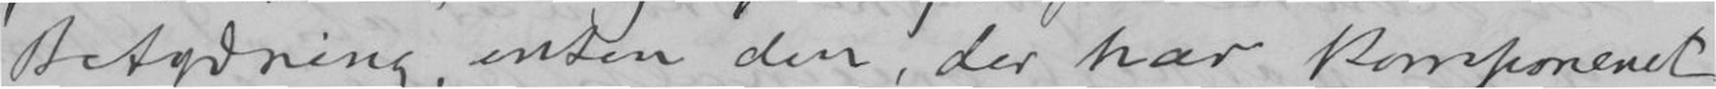Betydning, enten den, der har komponeret
